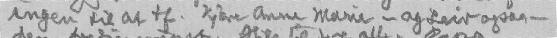ingen til at tf. Kjære Anne Marie - og Leiv ogsaa -
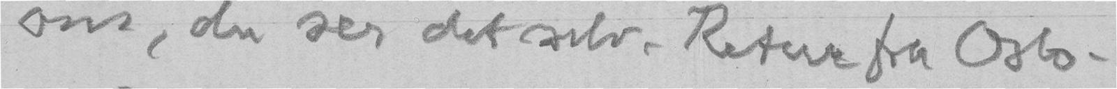om, du ser det selv. Retur fra Oslo.
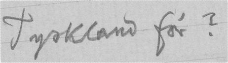Tyskland før?
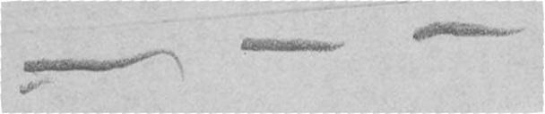- - -
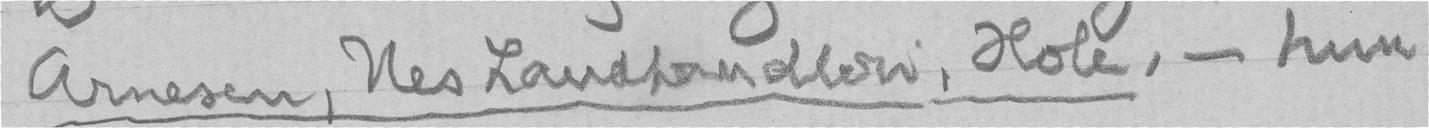Arnesen, Nes Landhandleri, Hole, - hun
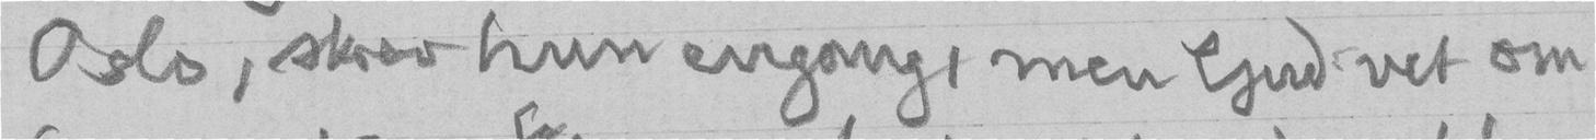Oslo, skrev hun engang, men Gud vet om
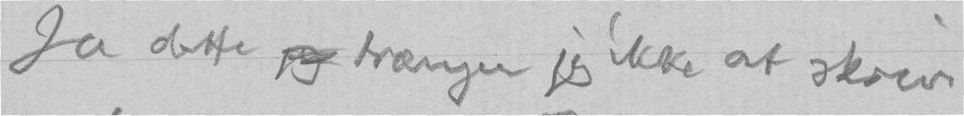Ja dette jeg trænger jeg ikke at skrive
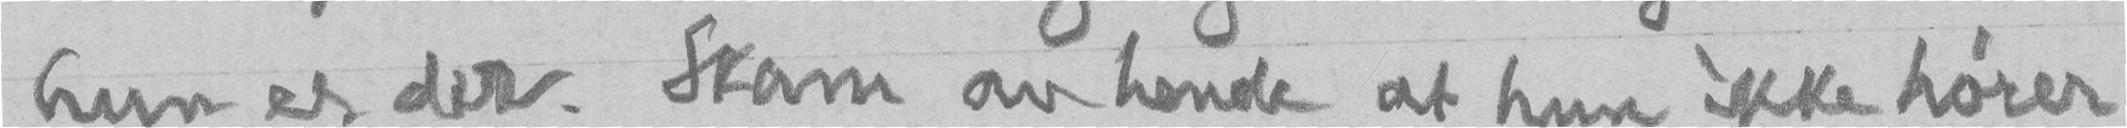hun er der. Skam av hende at hun ikke hører
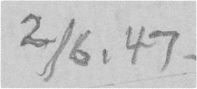2/6. 47.
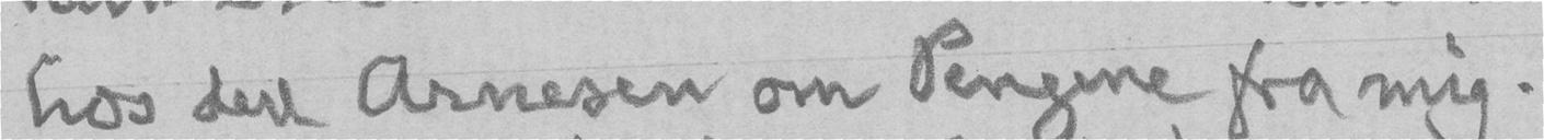hos den Arnesen om Pengene fra mig.
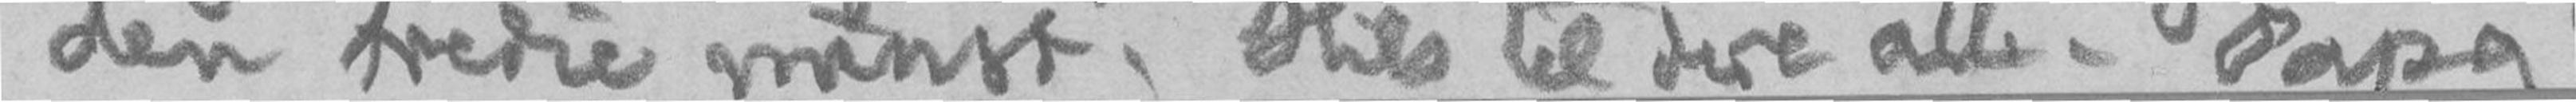den tredie minst. Hils til dere alle. Papa
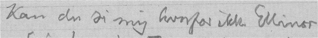Kan du si mig hvorfor ikke Ellinor
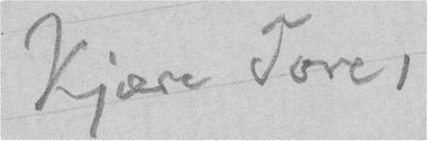Kjære Tore,
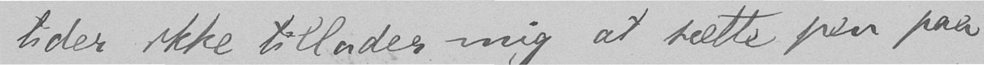tider ikke tillader mig at sætte pen paa
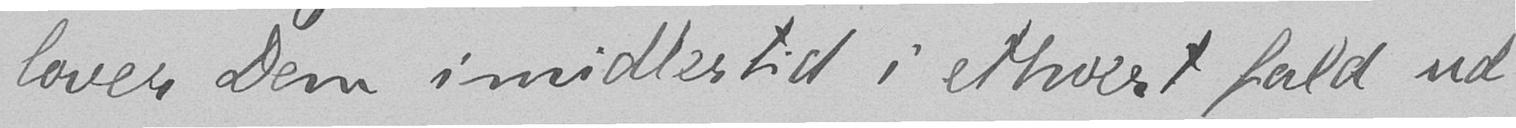lover Dem imidlertid i ethvert fald ud
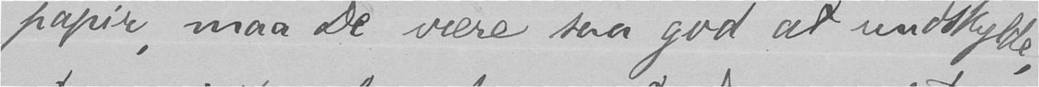papir, maa De være saa god at undskylde,
